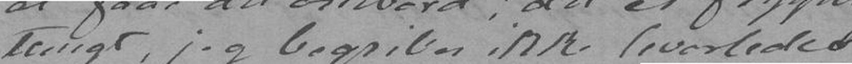tungt, jeg begriber ikke hvorledes
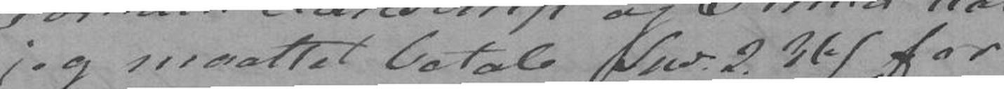jeg maattet betale Spd.2.36/ for
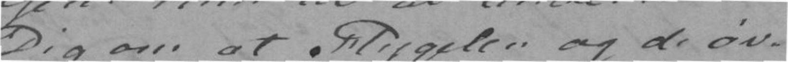Dig om at Flygelen og de øv-
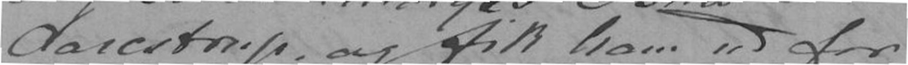Aarestrup, og fik ham ud for
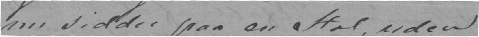nu sidder paa en Stol, uden
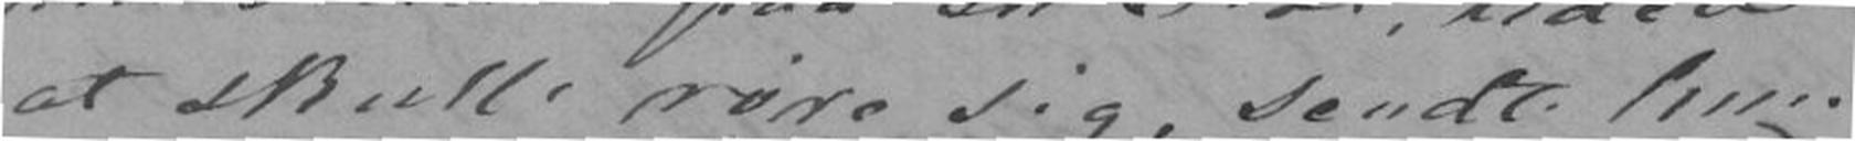at skulle røre sig, sendte hun
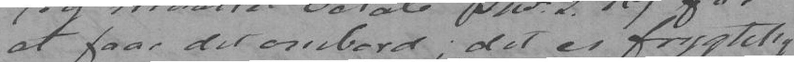at faae det ombord; det er frygtelig
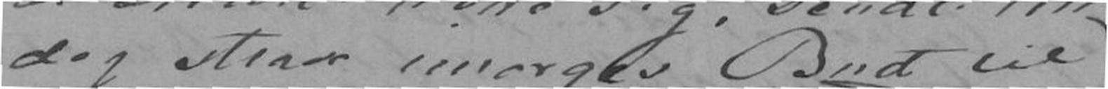dog strax imorges Bud til
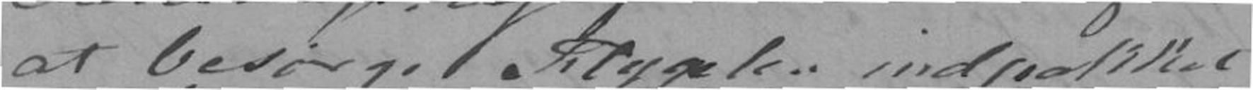at besørge Flygelen indpakket
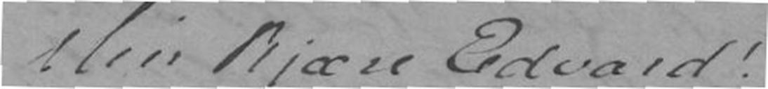Min kjære Edvard!
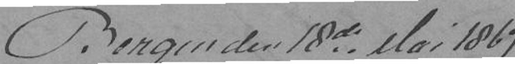Bergen den 18de Mai 1867.
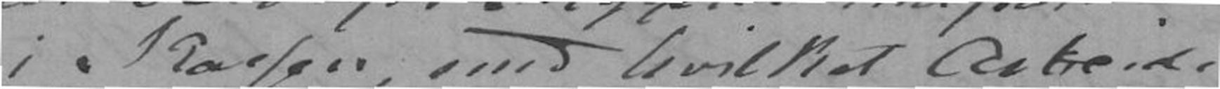i Kassen med hvilket Arbeide
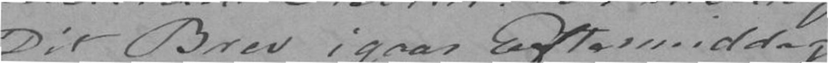Dig Brev igaar Eftermiddag
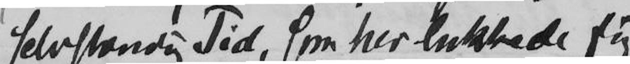selvstændig Tid, som her lukkede sig, -
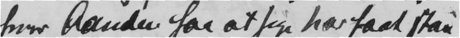hvor Aanden for at sige har faat staa
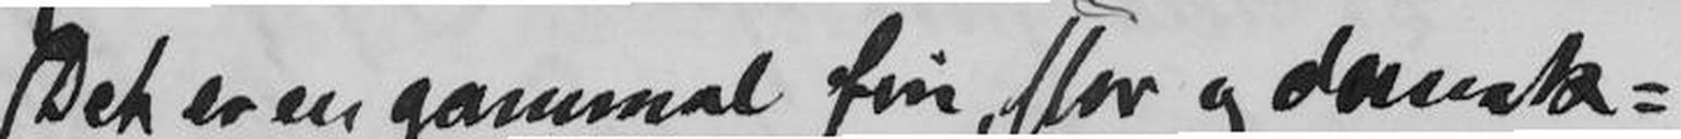Det er en gammel fin, stor og dansk-
